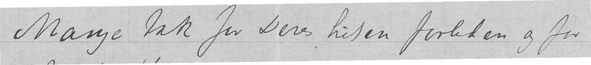Mange tak for Deres hilsen forleden og for
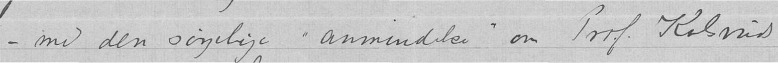– med den sørgelige "anmeldelse" om Prof. Kolsruds
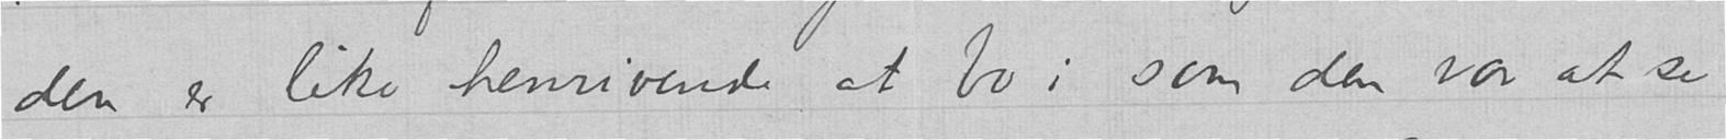den er like henrivende at bo i som den var at se
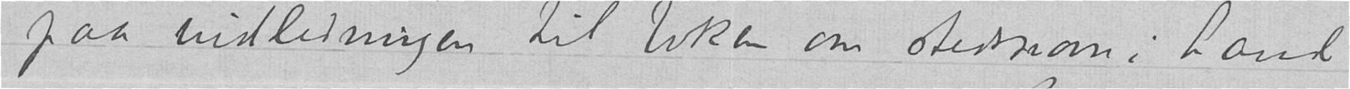paa indledningen til boken om stedsnavn i Land
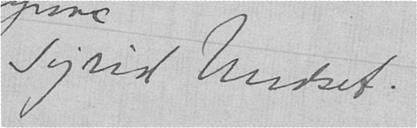Sigrid Undset.
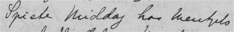Spiste Middag hos Wentzels
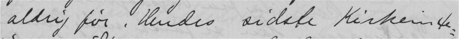aldrig før. Hendes sidste Kirkeinte-
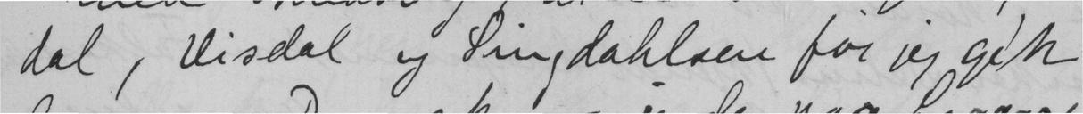dal, Visdal og Singdahlsen før jeg gik
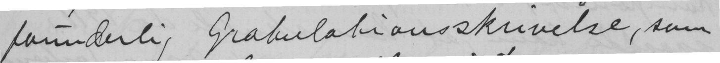forunderlig Gratulationsskrivelse, som
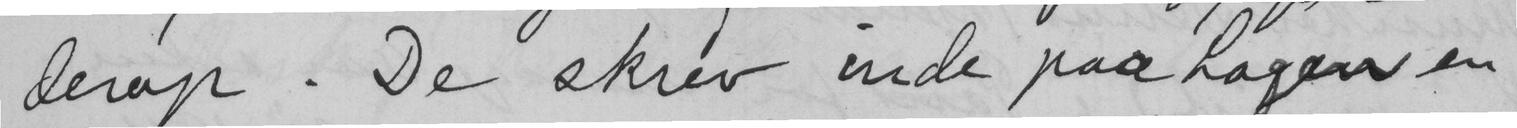derop. De skrev inde paa Logen en
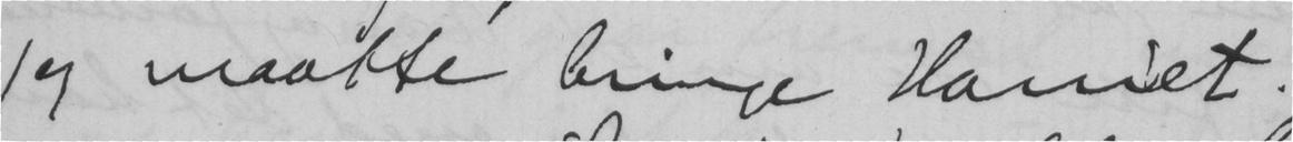jeg maatte bringe Harriet.
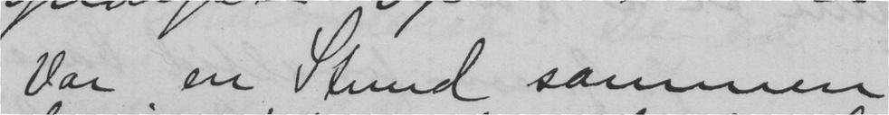Var en Stund sammen
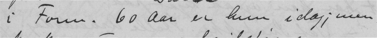i Form. 60 Aar er hun i dag; men
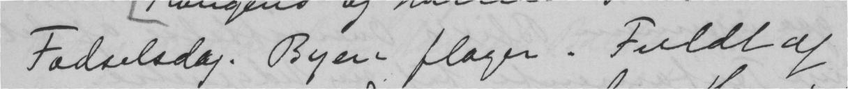Fødselsdag. Byen flager. Fuldt af
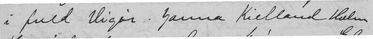i fuld Vigør. Janna Kielland Holm
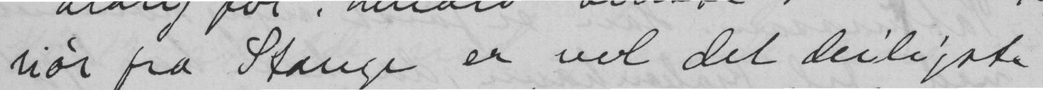riør fra Stange er vel det deiligste
